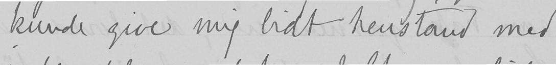kunde give mig lidt henstand med
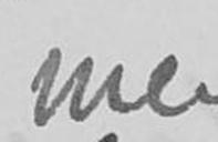men
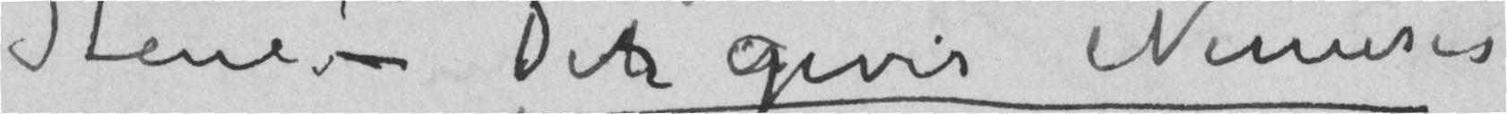Stene! – Detn giver Nemesis
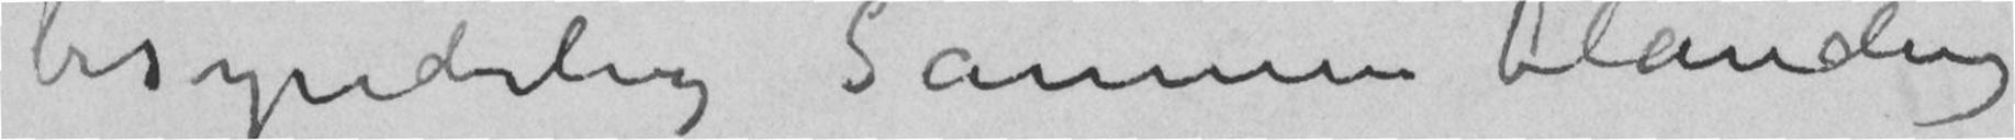besynderlig sammenblanding
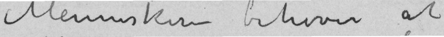Menneskerne behøver at
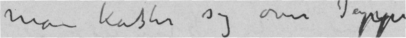man kaster sig over Jappe
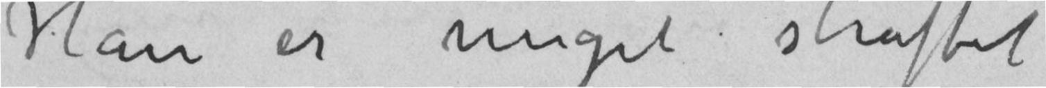Han er meget straffet
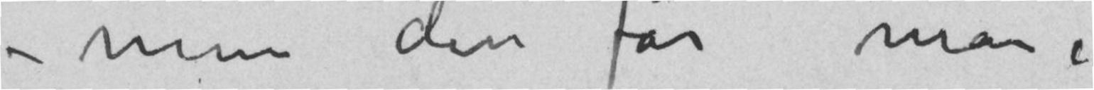– men den får man
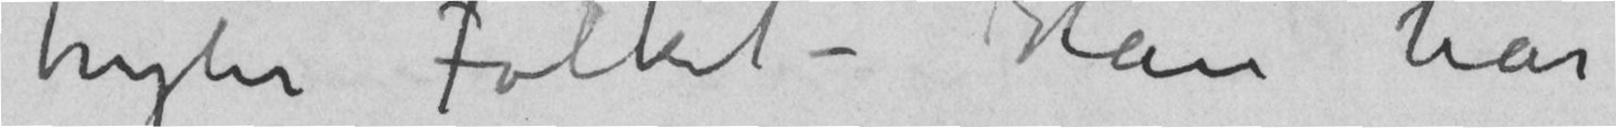hyler Folket – Han har
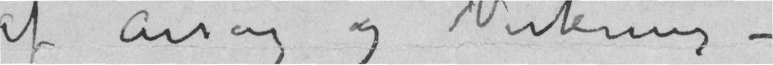af Årsag og Virkning –
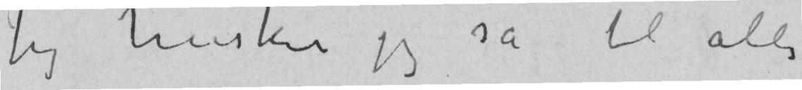Jeg husker jeg sa til alle
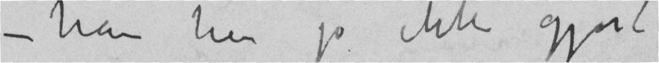– han har jo ikke gjort
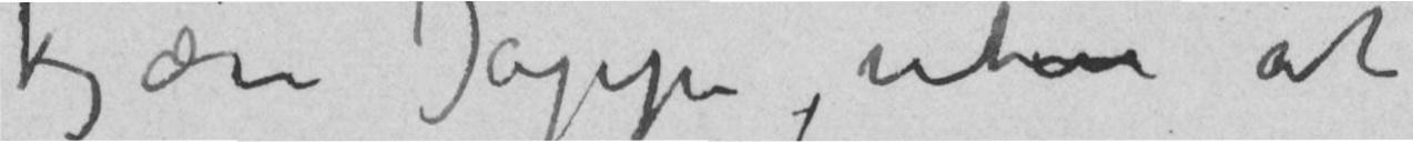kjære Jappe, uten at
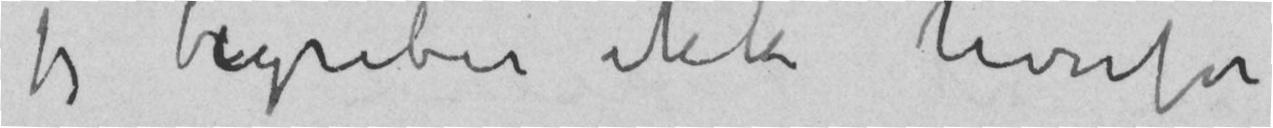jeg begriber ikke hvorfor
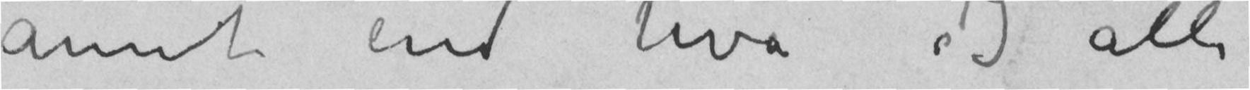annet end hva I alle
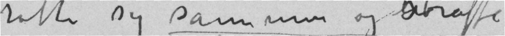rotte sig sammen og straffe
